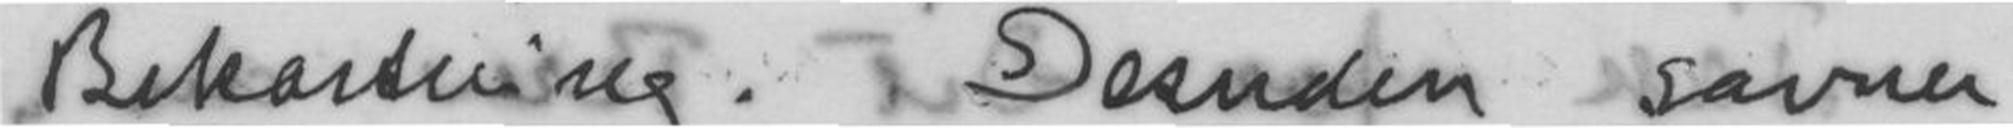Bekostning. Desuden savner
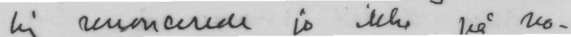lig renoncerede jo ikke på no-
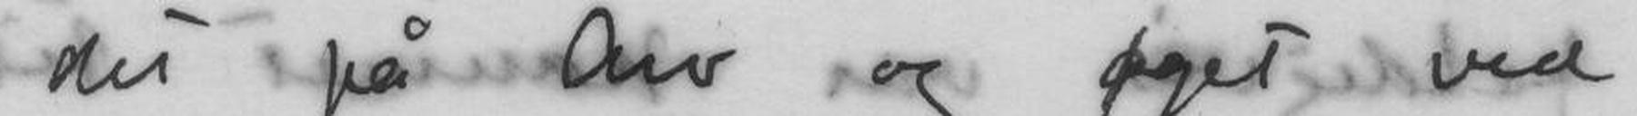det på Arv og øget ved
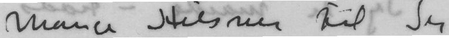Mange Hilsner til Jer
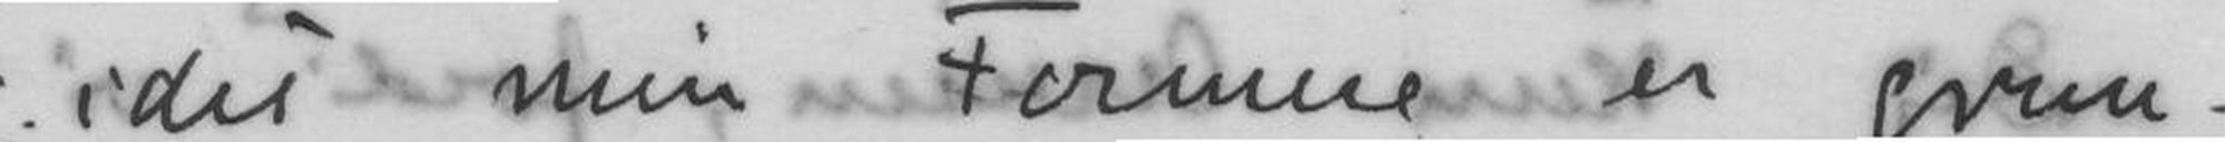i det min Formue er grun-
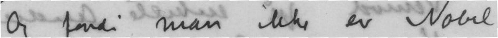Og fordi man ikke er Nobel
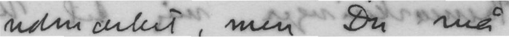udmærket, men Du må
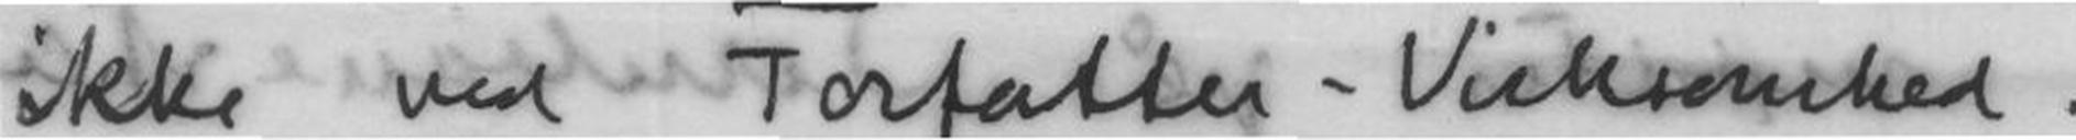ikke ved Forfatter Virksomhed.
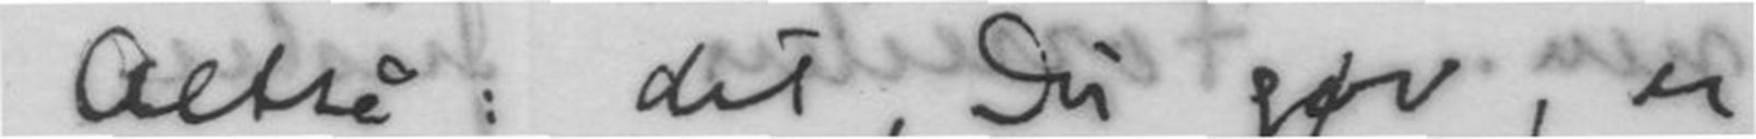Altså: det, Du gør; er
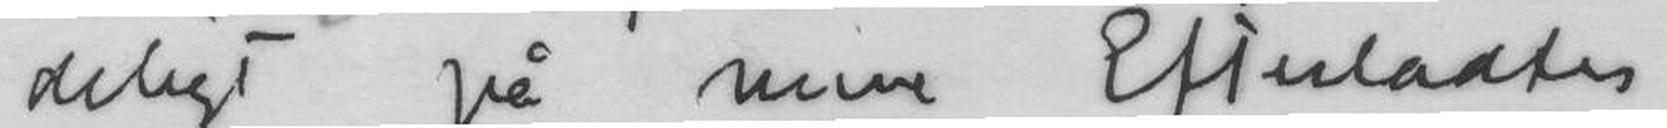deligt på mine Efterladtes
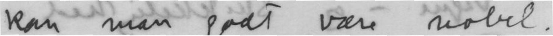kan man godt være nobel.
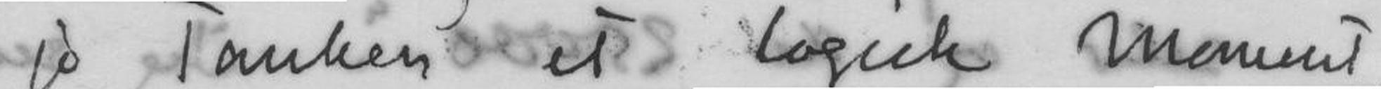jo Tanken et logisk Moment
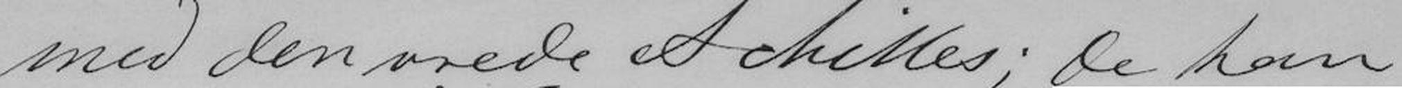med den vrede Achilles; De kan
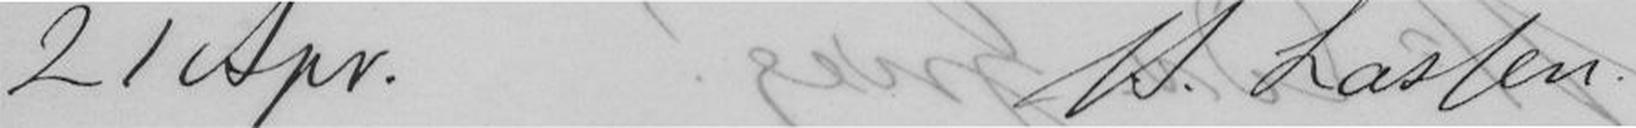21 April M. Lassen.
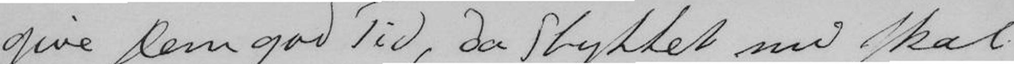give dem god Tid, da stykket nu skal
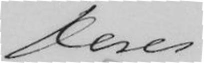Deres
